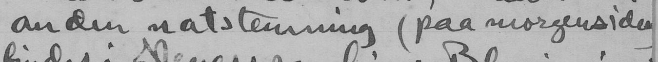anden natstemning (paa morgensiden
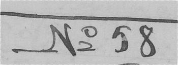No 58
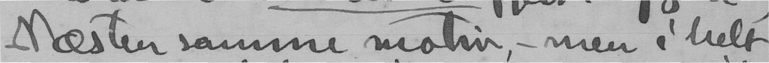Næsten samme motiv, - men i helt
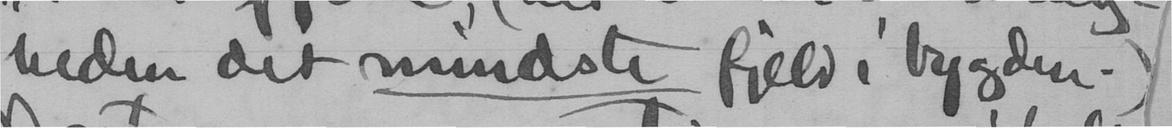heden det mindste fjeld i bygden.)
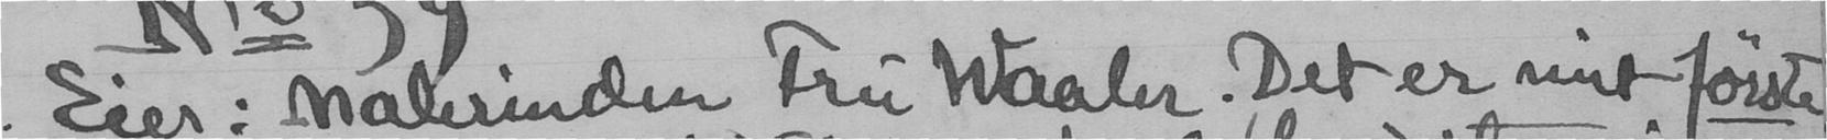Eier: malerinden Fru Waaler. Det er mit første
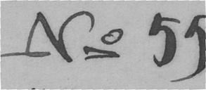No 55
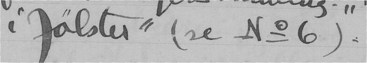i Jølster" (se No 6).
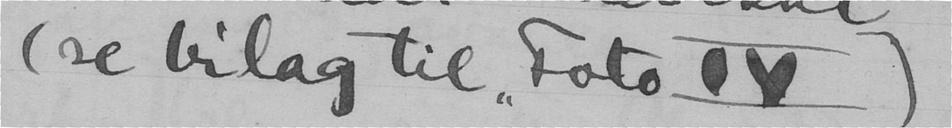(se bilag til "Foto IV)
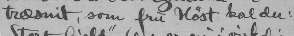træsnit, som fru Høst kalder:
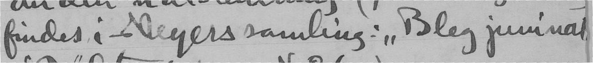findes i Meyers samling: "Bleg juninat
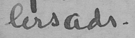lers adr.
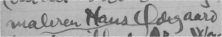maleren Hans Ødegaard
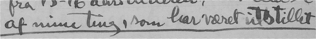af mine ting, som har været utstillet
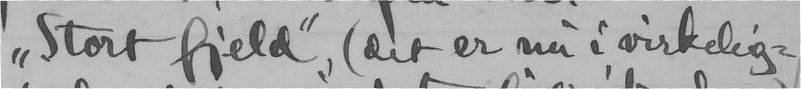"Stort fjeld", (det er nu i virkelig-
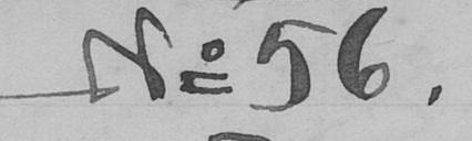No 56.
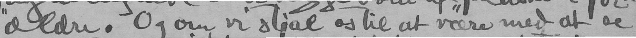ældre, Og om vi stjal os til at være med at se
